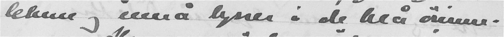lebene og ennå lyner i de blå øinene.
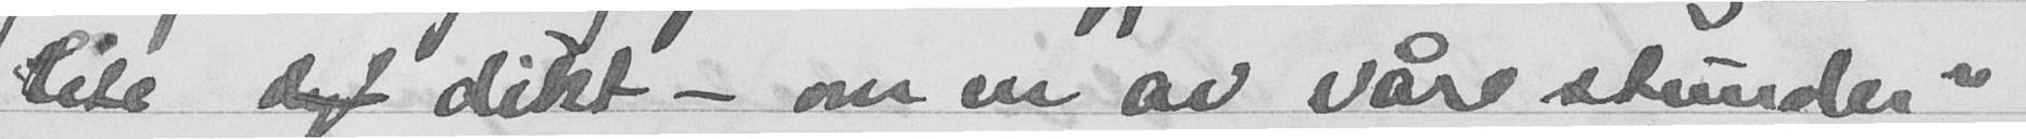lite  dikt - om en av "våre stunder"
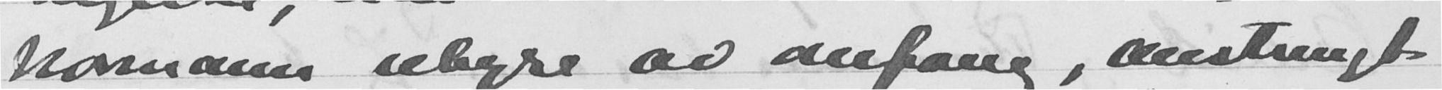Normann uhyre av omfang, anstrengt
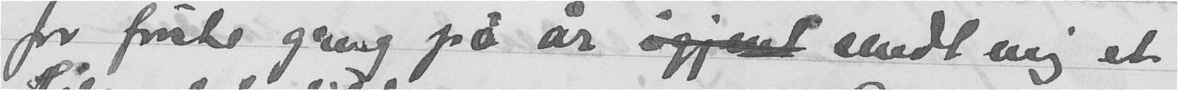for første gang på år  sendt mig et
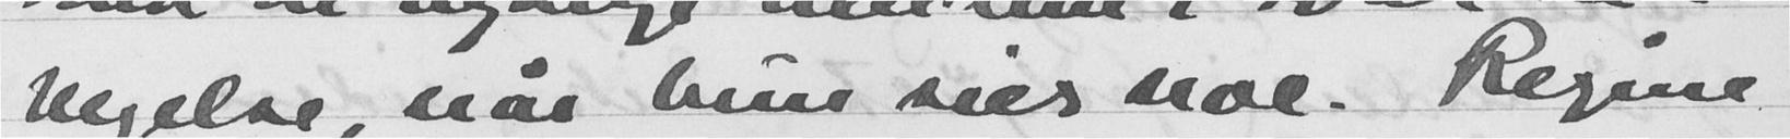vegelse, når hun sier noe. Regine
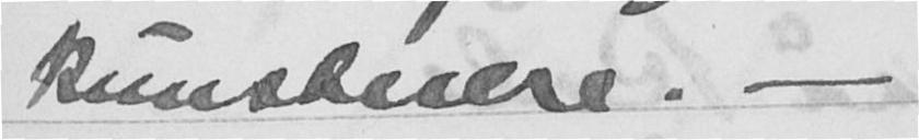kunstnere. -
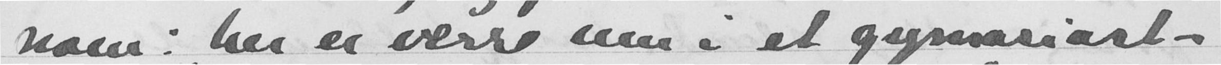man; "her er værre men i et gymasiast-
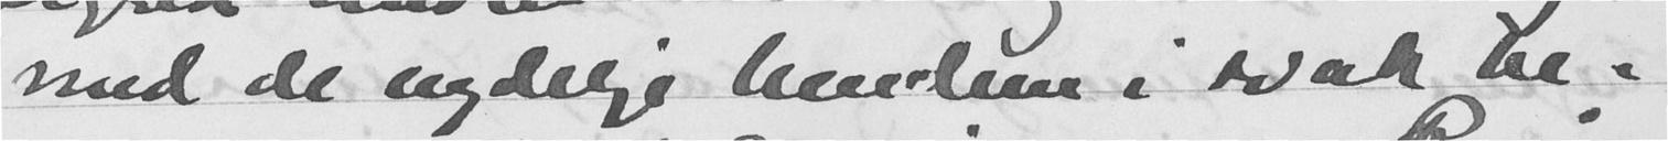med de nydelige hendene i svak be-
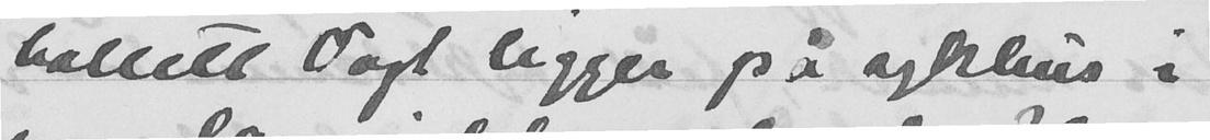Collett Vogt ligger på sykehus i
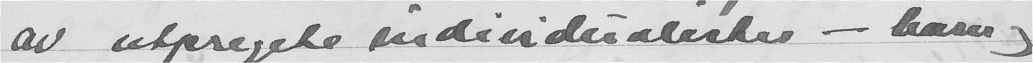av utpregete individualister - barn og
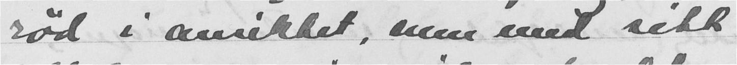rød i ansiktet, men med sitt
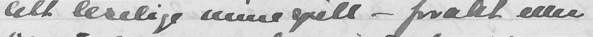lett leselige minespill - forakt eller
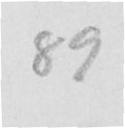89
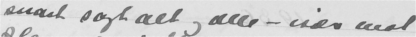snart sagt alt og alle - især mot
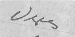Deres
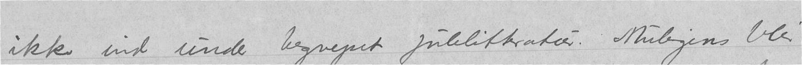ikke ind under begrepet julelitteratur. Muligens blir
